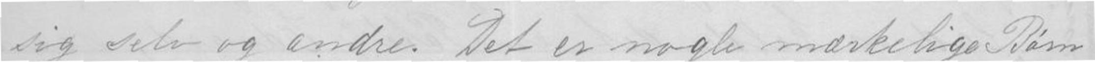sig selv og andre. Det er nogle mærkelige Børn
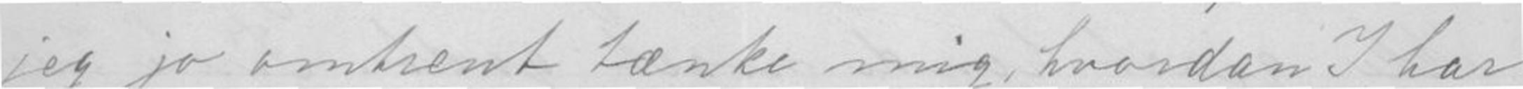jeg jo omtrent tænke mig, hvordan I har
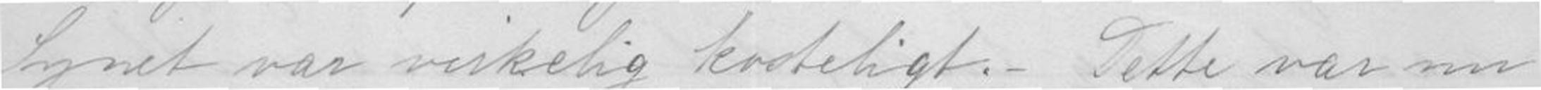Synet var virkelig kostelig.- Dette var nu
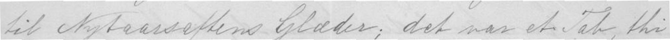til Nytaarsaftens Glæder; det var et Tab, thi
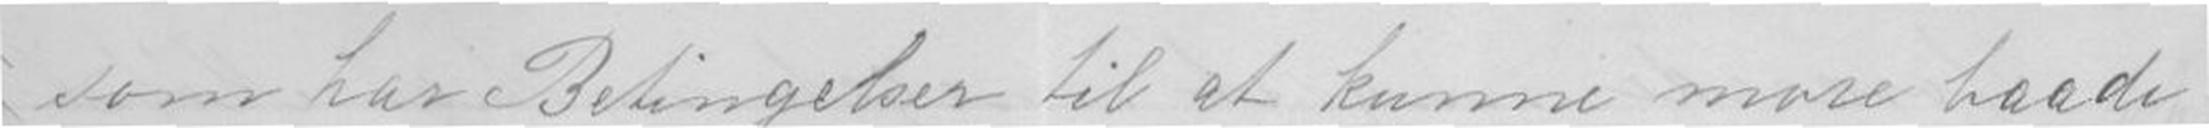som har Betingelser til at komme more baade
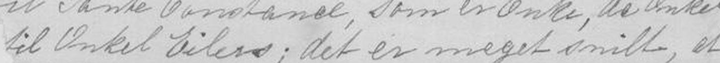til Onkel Eilers; det er meget snilt, at
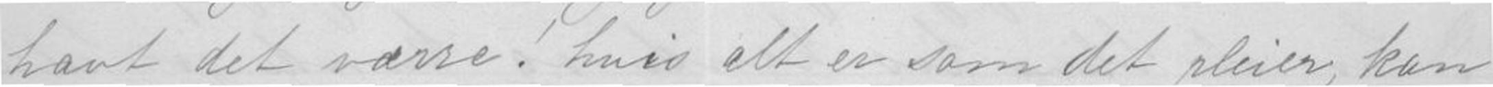havt det værre! hvis alt er som det pleier, kan
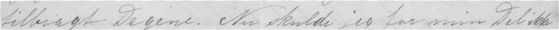tilbragt Dagene. Nu skulde jeg for min Del ikke
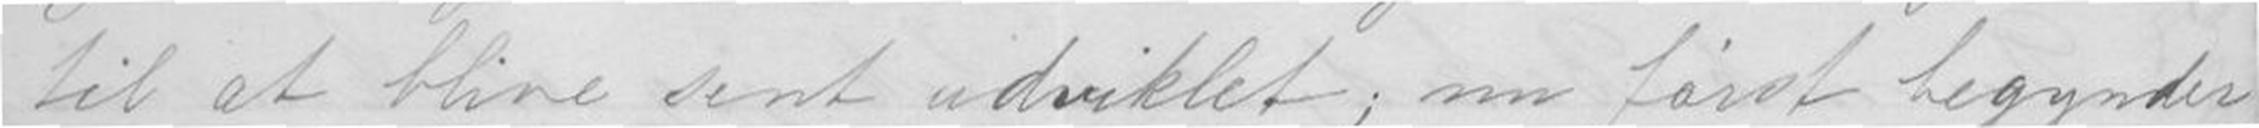til at blive sent udviklet; nu først begynder
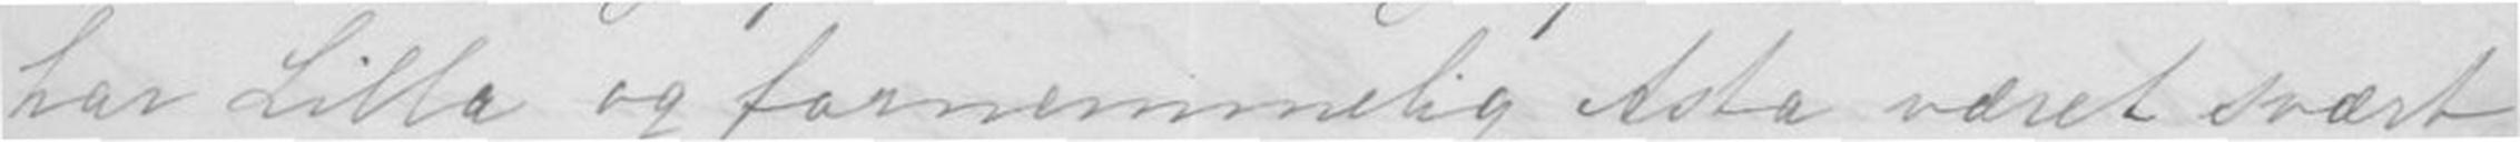har Lilla og fornemmelig Asta været svært
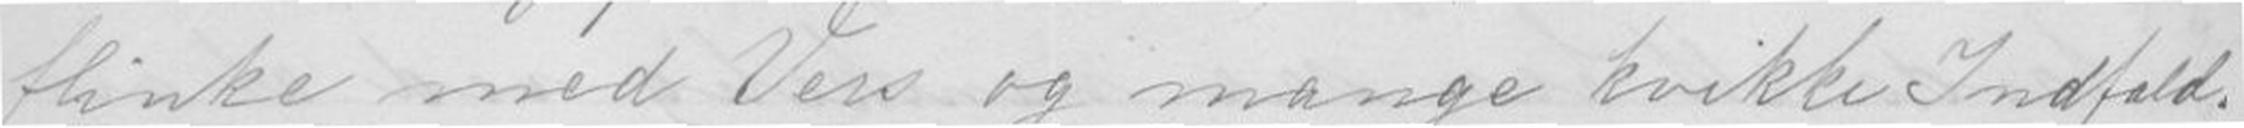flinke med Vers og mange kvikke Indfald.
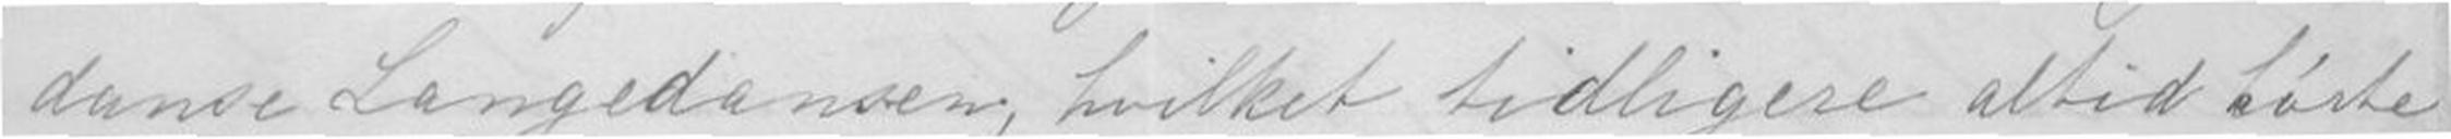danse Langedansen, hvilket tidligere altid bøte
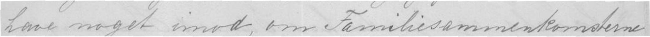have noget imod, om Familiesammenkomstene
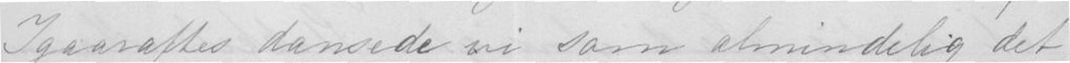Igaaraftens dansede vi som almindelig det
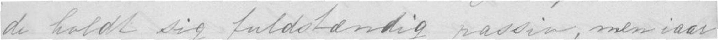de holdt sig fuldstændig passiv, men iaar
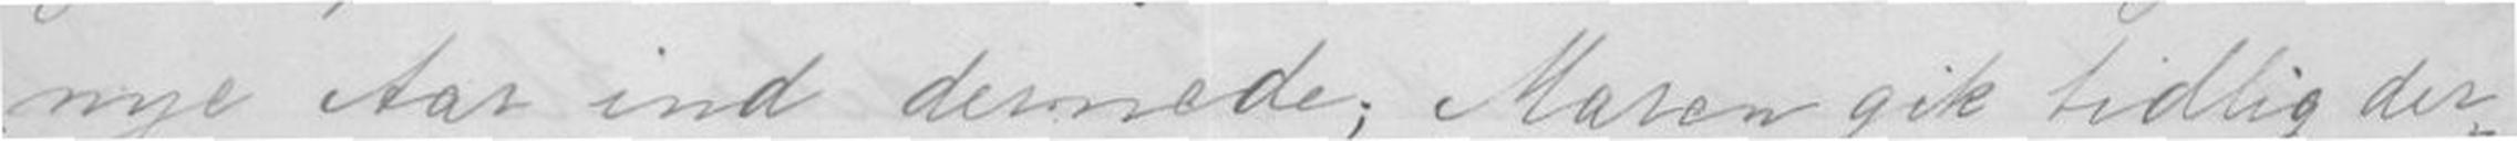nye Aar ind dermede; Maren gik tidlig der-
